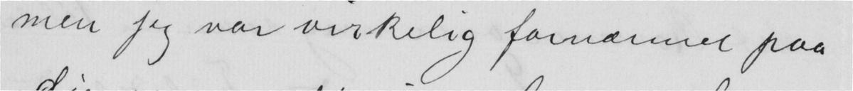men jeg var virkelig fornærmet paa
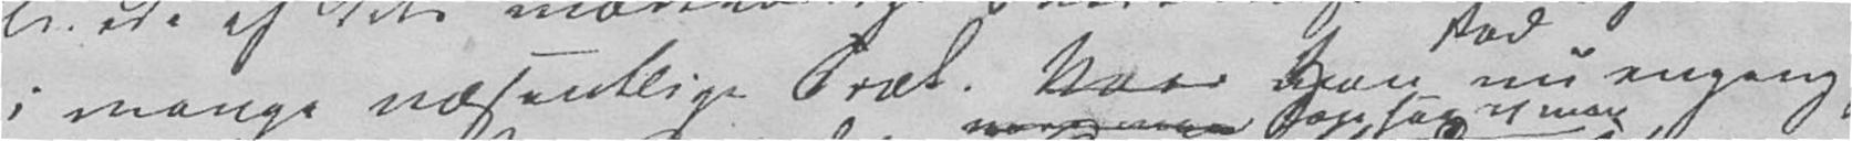i mange væsentlige Træk. Naar Han nu engang
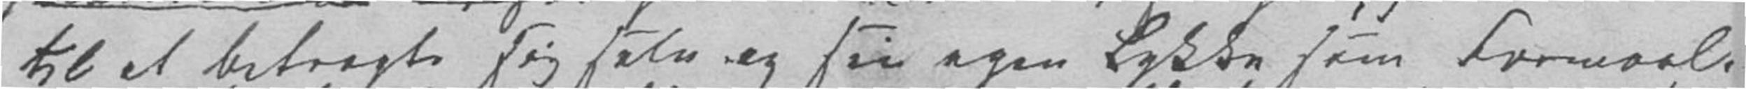til at betragte sig selv og sin egen Lykke som Formaal,
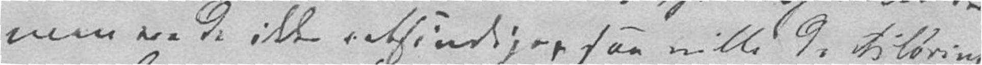men ere de ikke retsindige, saa ville de tilbringe
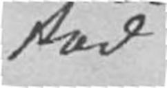stod
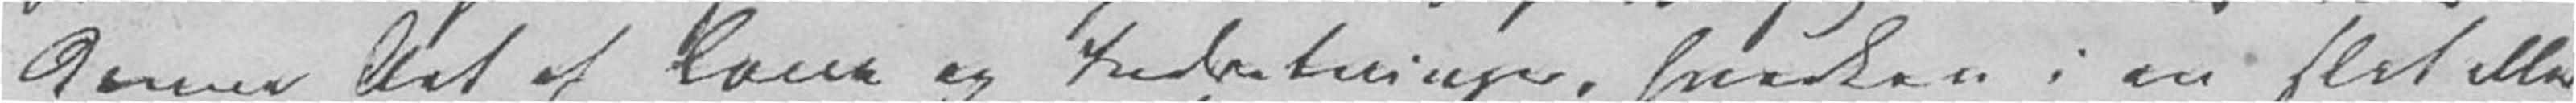denne Art af Love og Indretninger, hverken i en slet eller
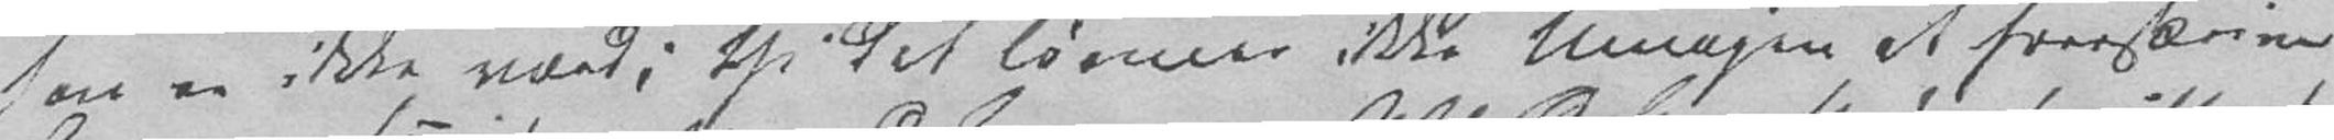han er ikke værd; thi det lønner ikke Umagen at foreskrive
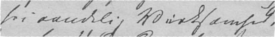uden fri aandelig Virksomhed,
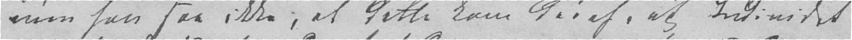men han saa ikke, at dette kom deraf, at Individet
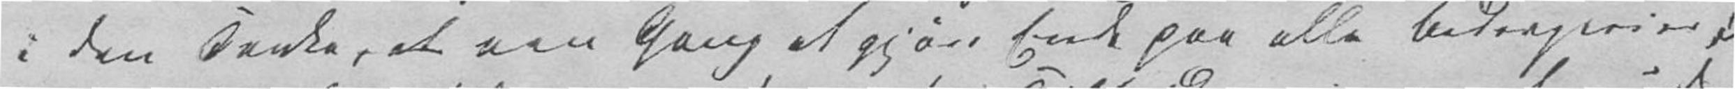i den Tanke, at een Gang at gjøre Ende paa alle Bedragerier i
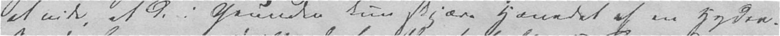at vide, at de i Grunden kun skjære Hovedet af en Hydra.
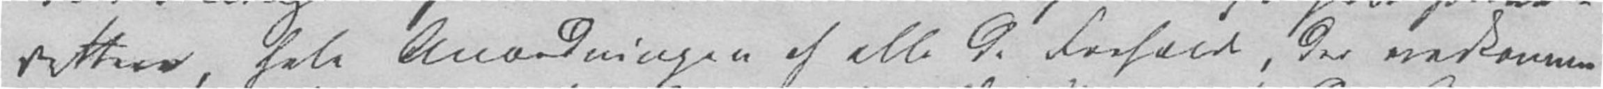retten, hele Anordningen af alle de Forholde, der vedkommer
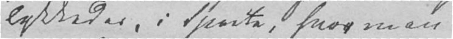lykkedes, i Sparta, hvor man
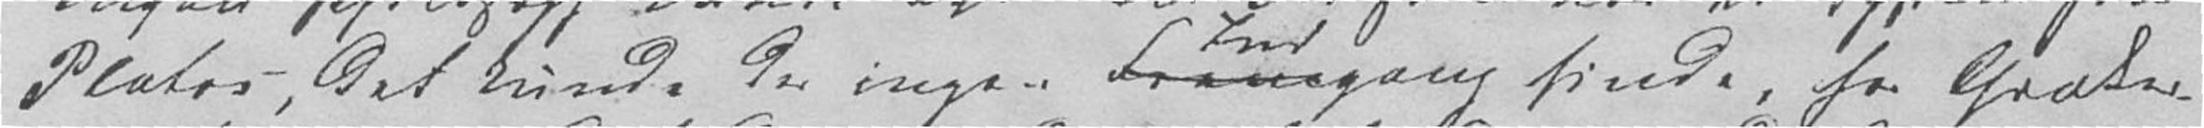Platos, det kunde der ingen Fremgang finde, hos Græker-
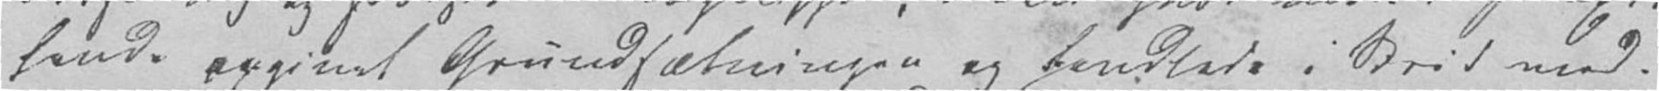havde opgivet Grundsætningen og handlede i Strid med.
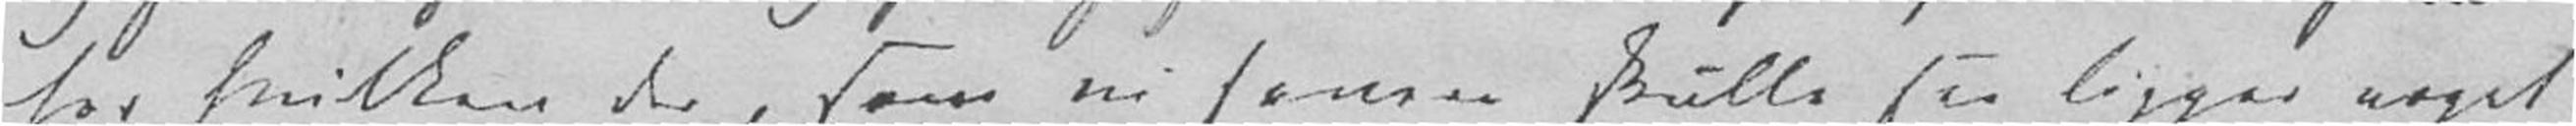for hvilken der, som vi senere skulle see ligger noget
